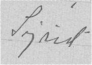Sigrid
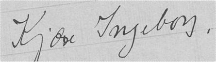Kjære Ingeborg,
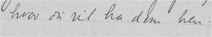hvor du vil ha dem hen.
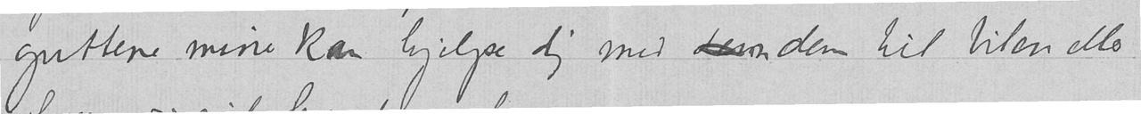guttene mine kan hjelpe dig med dem dem til bilen eller
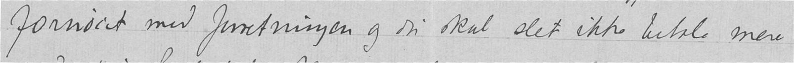fornøiet med forretningen og du skal slet ikke betale mere
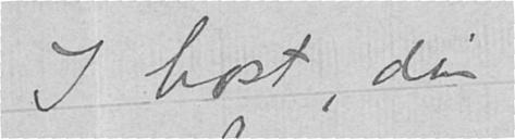I hast, din
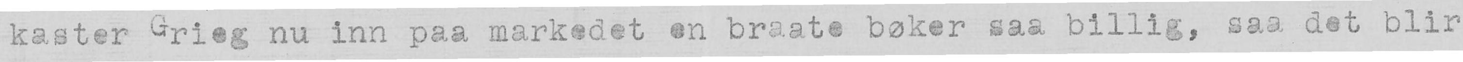kaster Grieg nu inn paa markedet en braate bøker saa billig, saa det blir
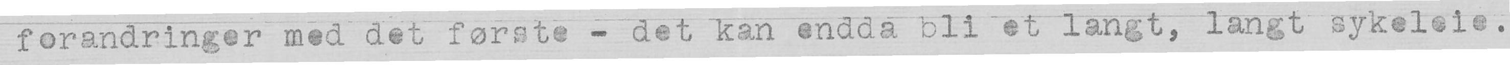forandringer med det forste - det kan endda bli et langt, langt sykeleie.
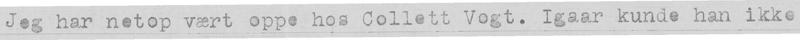Jeg har netop vært oppe hos Collett Vogt. Igaar kunde han ikke
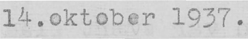14.oktober 1937.
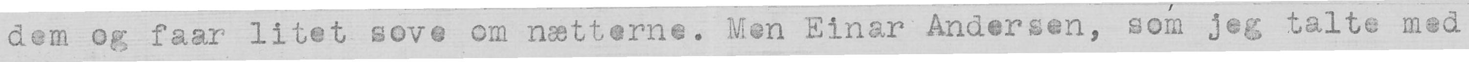dem og faar litet sove om nætterne. Men Einar Andersen, som jeg talte med
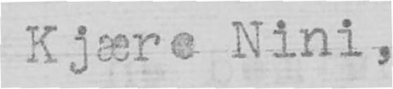Kjære Nini,
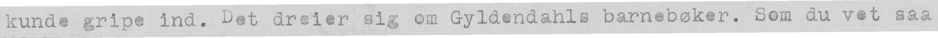kunde gripe ind. Det dreier sig om Gyldendahls barnebøker. Som du vet saa
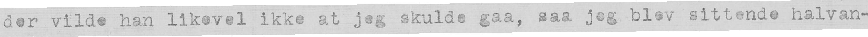der vilde han likevel ikke at jeg skulde gaa, saa jeg blev sittende halvan-
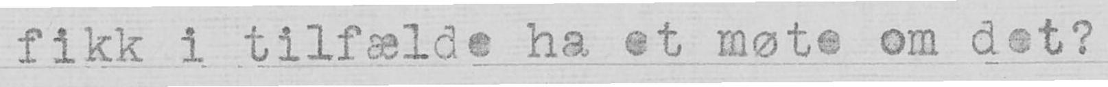fikk i tilfælde ha et møte om det?
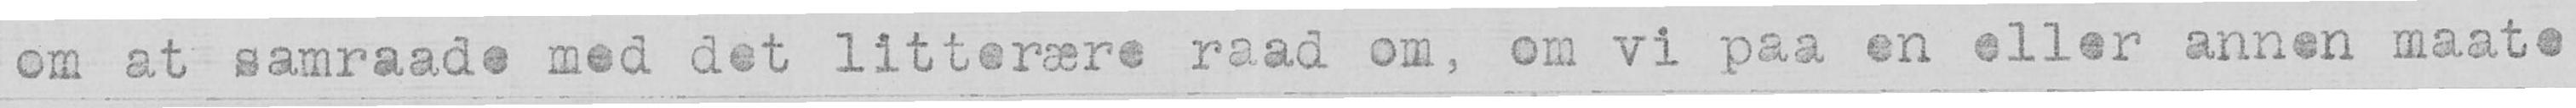om at samraade med det litterære raad om, om vi paa en eller annen maate
